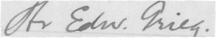Hr. Edw. Grieg.
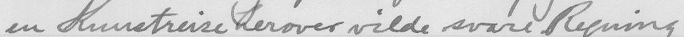en Kunstreise herover vilde svare Regning
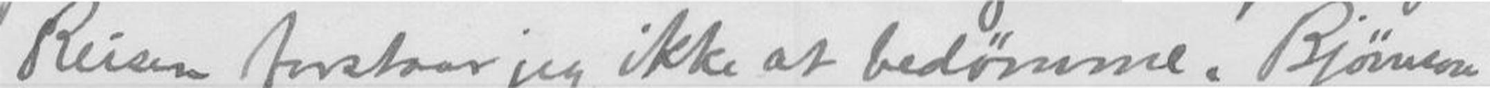Reisen forstaar jeg ikke at bedømme. Bjørnson
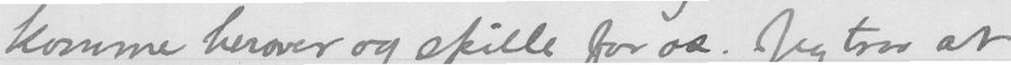komme herover og spille for os. Jeg tror at
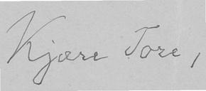Kjære Tore,
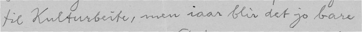til Kulturbeite, men iaar blir det jo bare
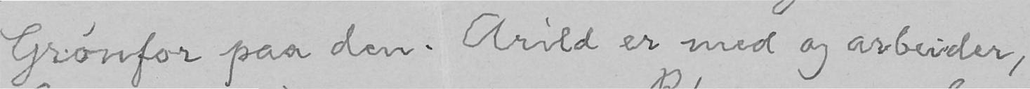Grønfor paa den. Arild er med og arbeider,
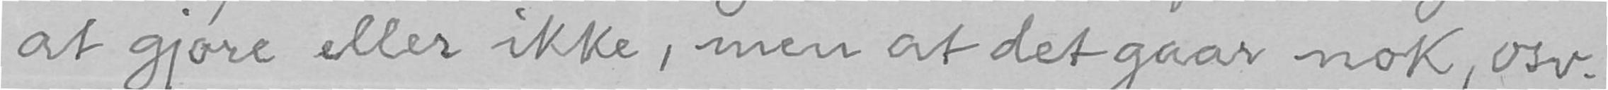at gjøre eller ikke, men at det gaar nok, osv.
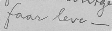faar leve -
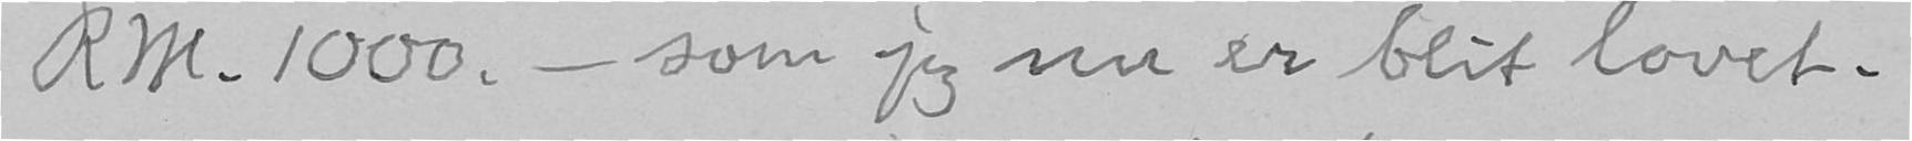RM. 1000. - som jeg nu er blit lovet.
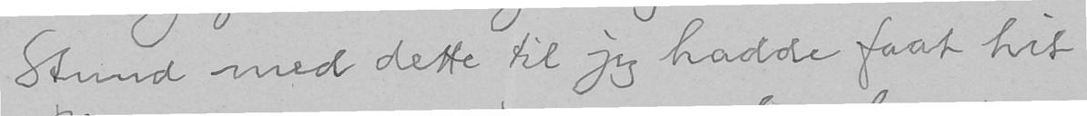Stund med dette til jeg hadde faat hit
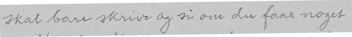skal bare skrive og si om du faar noget
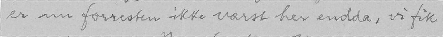er nu forresten ikke værst her endda, vi fik
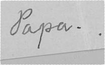Papa.
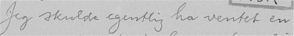Jeg skulde egentlig ha ventet en
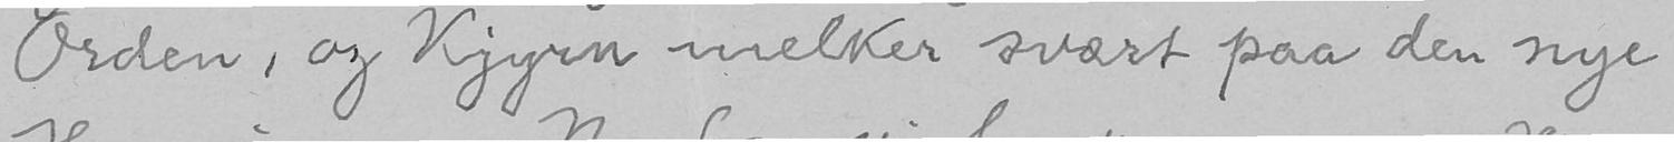Orden, og Kjyrn melker svært paa den nye
